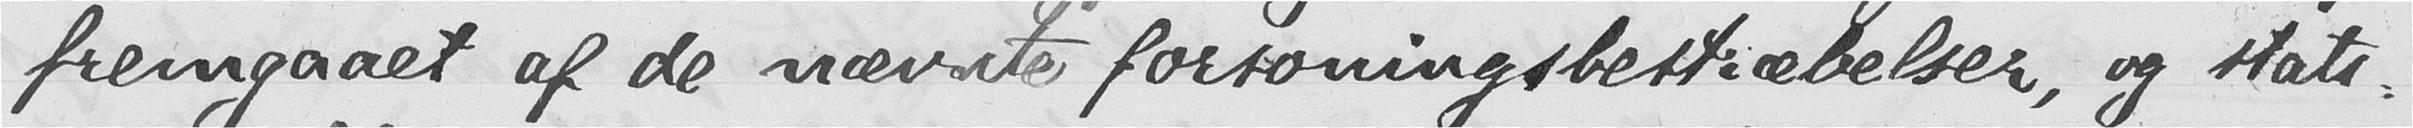fremgaaet af de nævnte forsoningsbestræbelser, og stats-
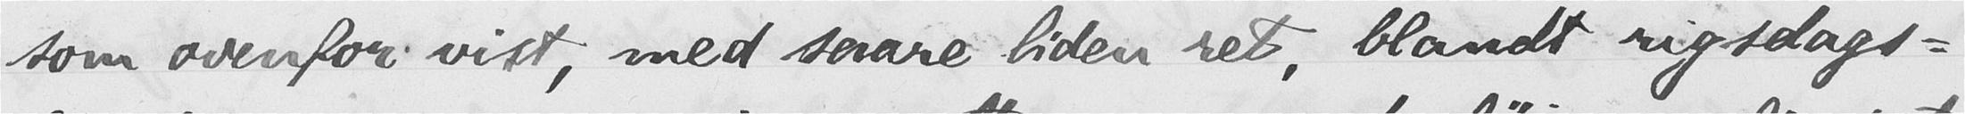som ovenfor vist, med saare liden ret, blant rigsdags-
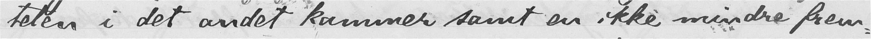teten i det andet kammer samt en ikke mindre frem-
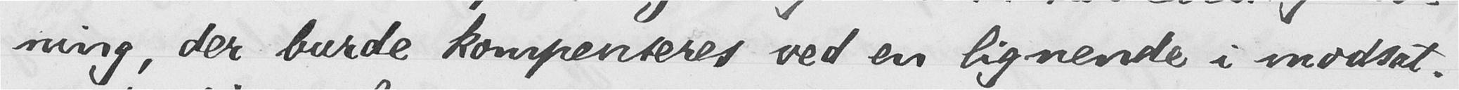ning, der burde kompenseres ved en lignende i modsat.
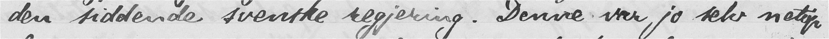den siddende svenske regjering. Denne var jo selv netop
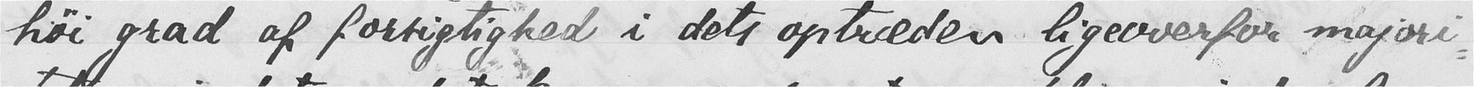høi grad af forsigtighed i dets optræden ligeoverfor majori-
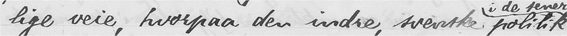lige veie, hvorpaa den indre, svenske politik
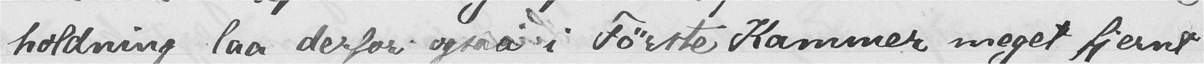holdning laa derfor ogsaa i Første Kammer meget fjernt
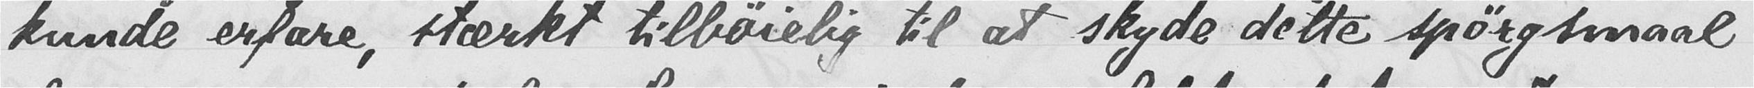kunde erfare, stærkt tilbøielig til at skyde dette spørgsmaal
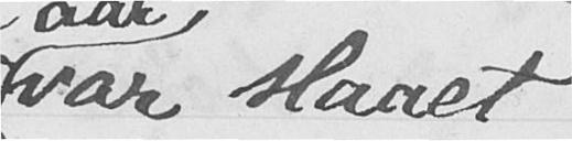var slaaet
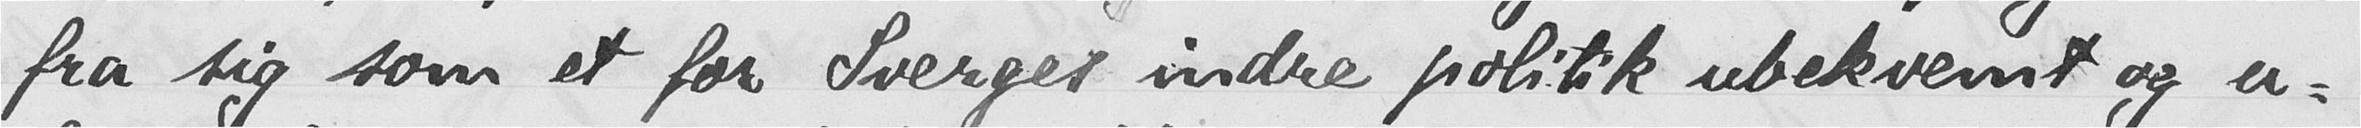fra sig som et for Sverges indre politik ubekvemt og u-
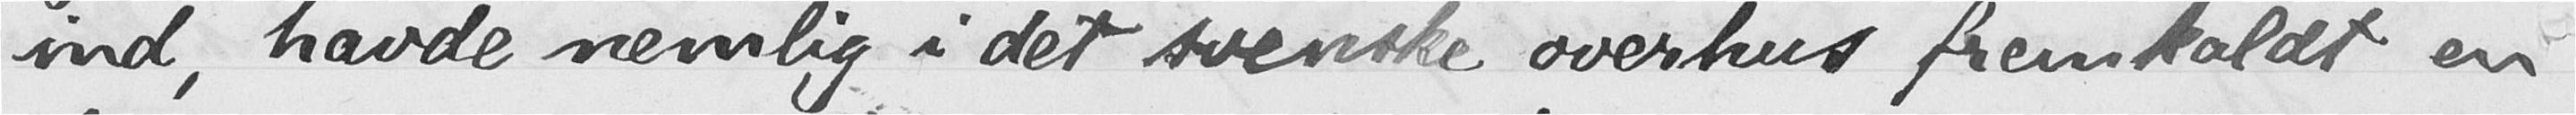ind, havde nemlig i det svenske overhus fremkaldt en
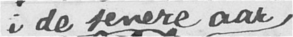i de senere aar
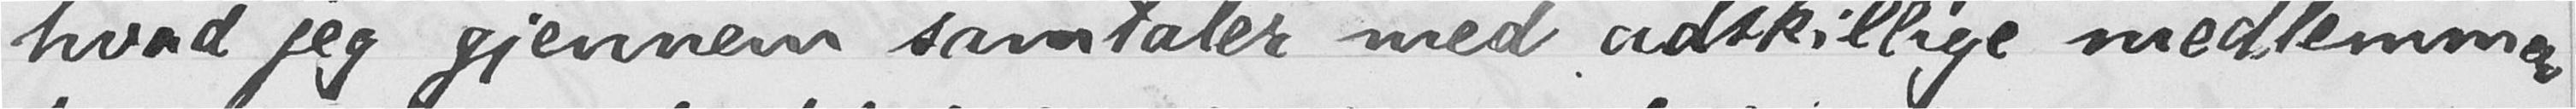hvad jeg gjennem samtaler med adskillige medlemmer
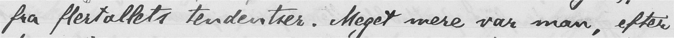fra flertallets tendentser. Meget mere var man, efter
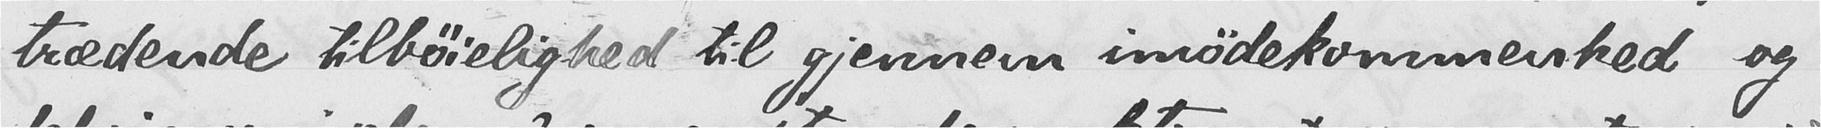trædende tilbøielighed til gjennem imødekommenhed og
